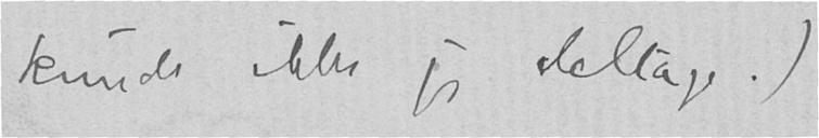kunde ikke jeg deltage.)
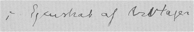i Egenskab af Arvtager
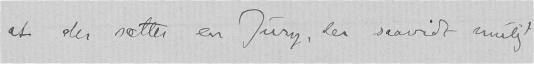at der sættes en Jury, der saavidt muligt
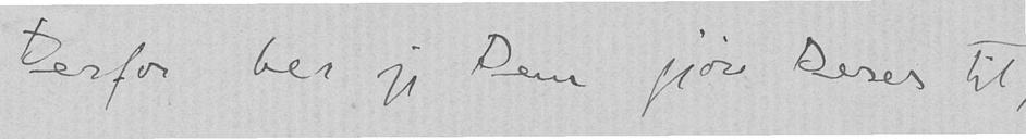Derfor ber jeg Dem gjøre Deres til,
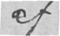af
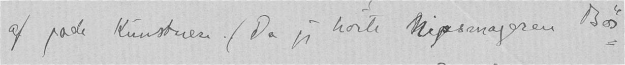af gode Kunstnere. (Da jeg hørte Nipsmageren Bø-
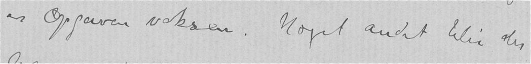er Opgaven voksen. Noget andet blir der
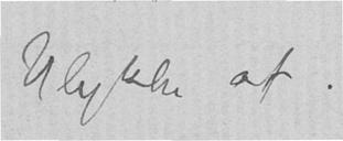Ulykke af.
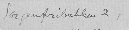Sorgenfribakken 2,
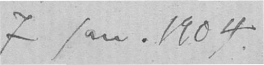7 Jan. 1904
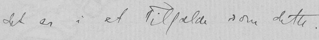det er i et Tilfælde som dette.
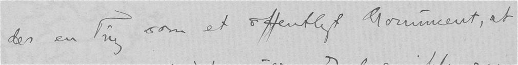der en Ting som et offentligt monument, at
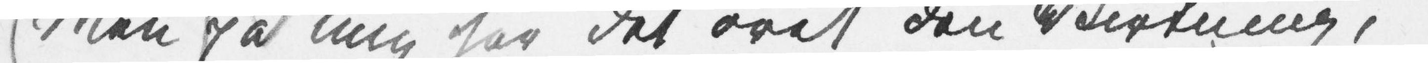Men på mig har det øvet den Virkning,
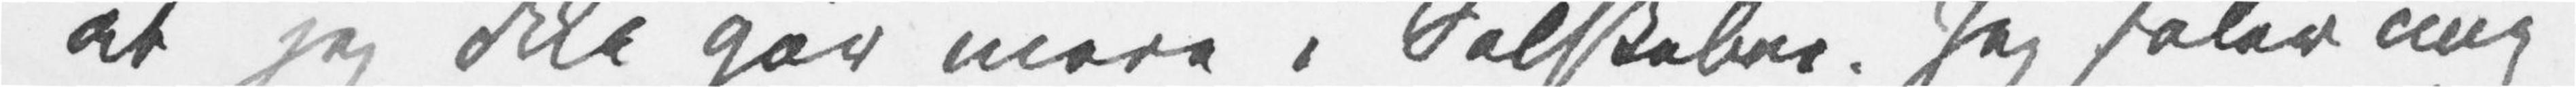at jeg ikke går mere i Selskaber. Jeg føler mig
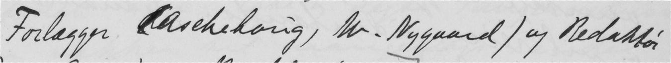Forlægger (Aschehoug, W. Nygaard) og Redaktør
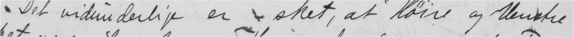Det vidunderlige er sket, at Høire og Venstre
